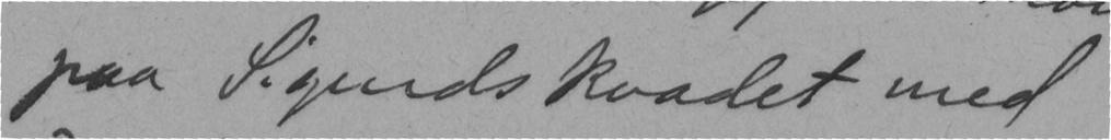paa Sigurds kvadet med
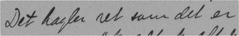Det hagler ret som det er
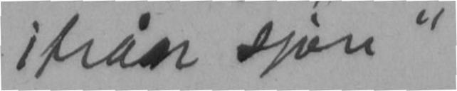ifrån sjøn"
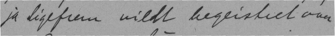ja ligefrem vildt begeistiet over
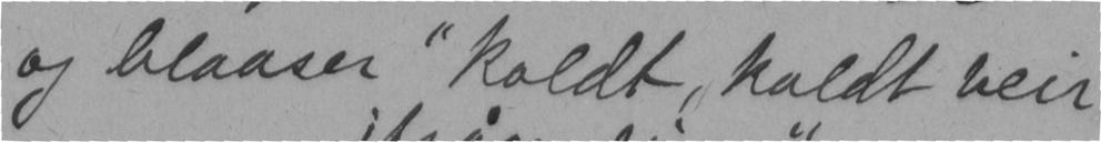og blaaser "kaldt, kaldt veir
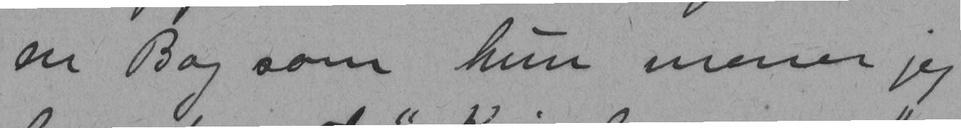en Bog som hun mener jeg
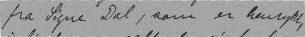fra Signe Dal, som er henrykt,
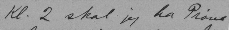Kl. 2 skal jeg ha Prøve
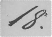18.
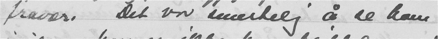fravær. Det var smertelig å se ham
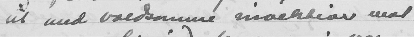ut med voldsomme motaktion mot
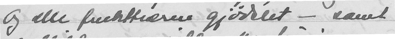Og alle frukttrærne gjødslet - samt
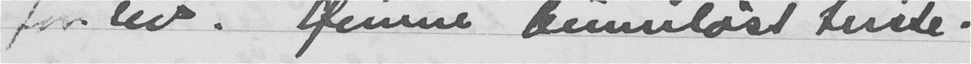for liv. Øinene bunnløst triste.
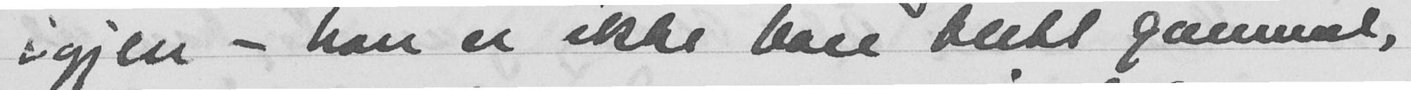igjen - han er ikke bare blitt gammel,
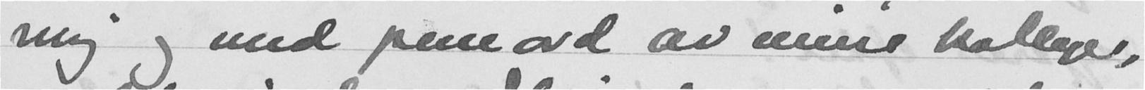mig og med pene ord av mine kolleger,
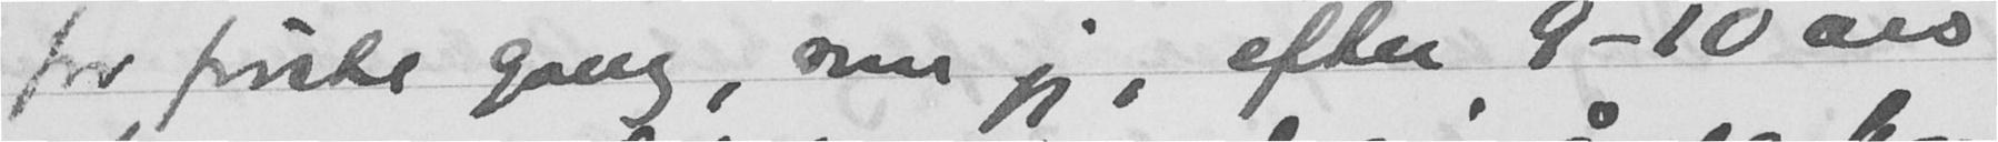for første gang, som jeg, efter 9 - 10 års
